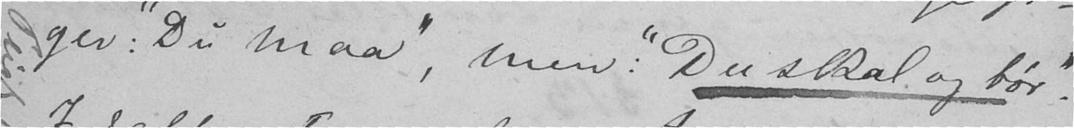ger: "Du maa", men: "Du skal og bør".
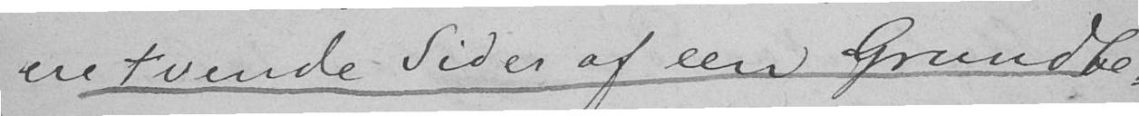ere tvende Side af een Grundbe-
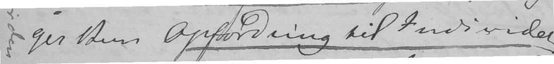ger kun Opfordring til Individet
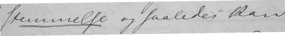stemmelse og saaledes kan
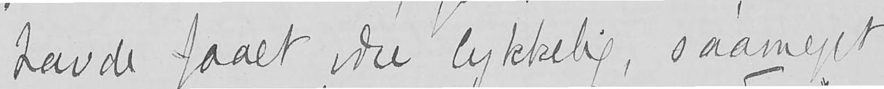havde faaet være lykkelig, saameget
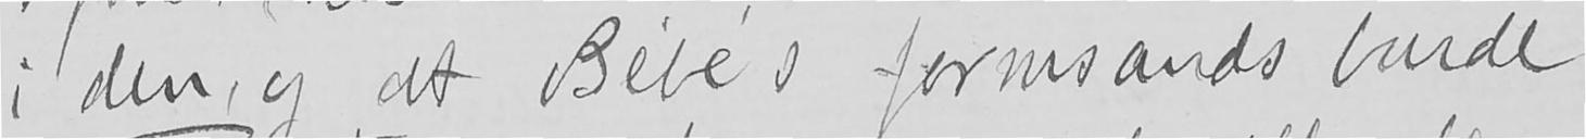i den, og at Bebes formsands burde
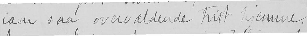iaar saa overvældende trist hjemme.
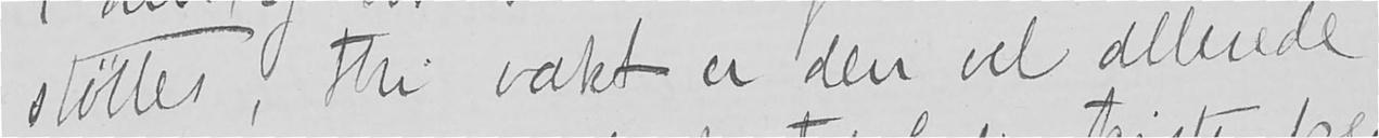støttes, thi vakt er den vel allerede.
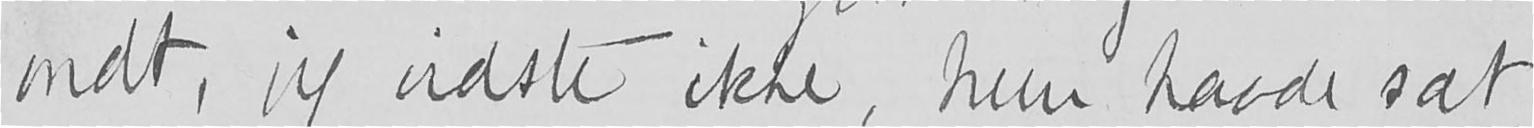ondt, jeg vidste ikke, hun havde sat
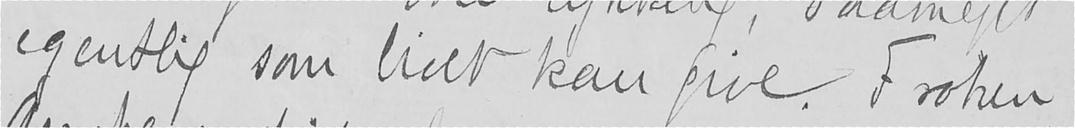egentlig som livet kan give. Frøken
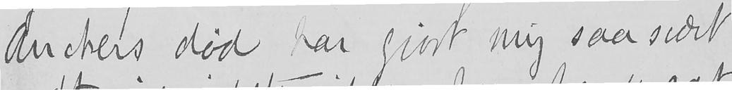Anchers død har gjort mig saa svært
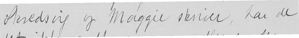Skredsvig og Maggie skriver, har de
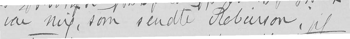var mig, som sendte Robinson, jeg
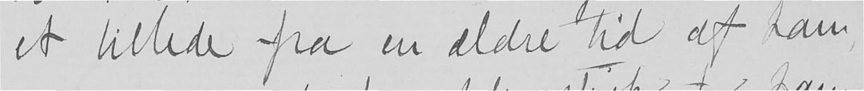et billede fra en ældre tid af ham,
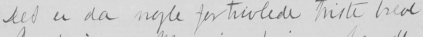Det er da nogle fortvivlede triste breve
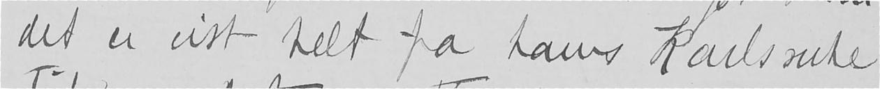det er vist helt fra hans Karlsruhe
